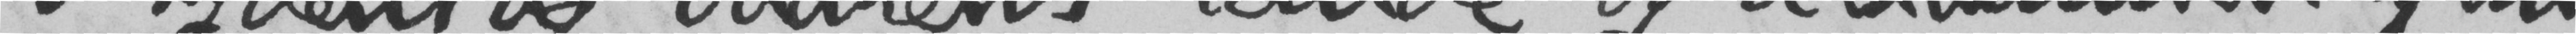i sydens og vaarens lande og velkommen hjem
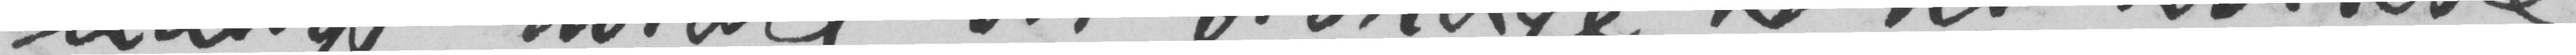udsigt, hvilket vil bidrage til at kvikke
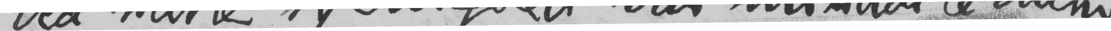Ved sidste stjernefald var mirabile dictu
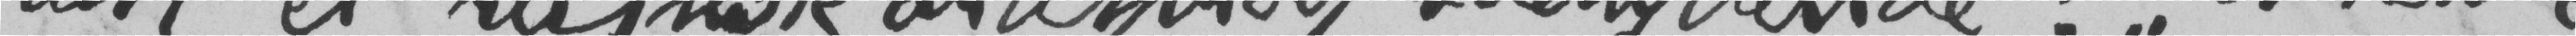lært et russisk ordsprog saalydende: «Vi hente
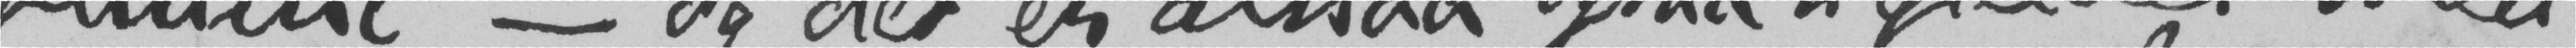fluunt,» – og det er altsaa ogsaa tilfældet med
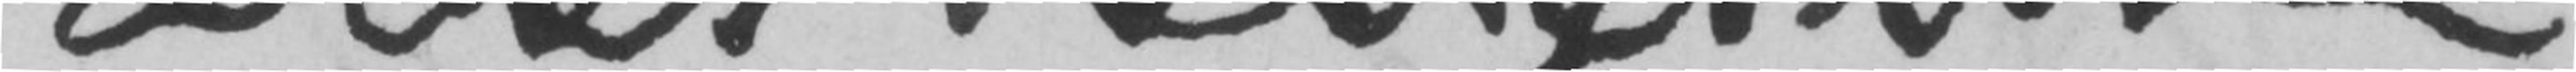Deres hengivne
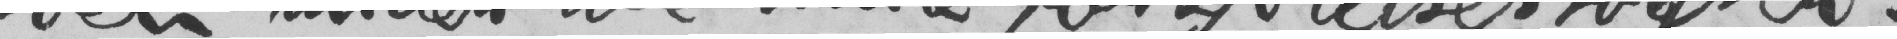ven under alle mine forkjølelsestogter.
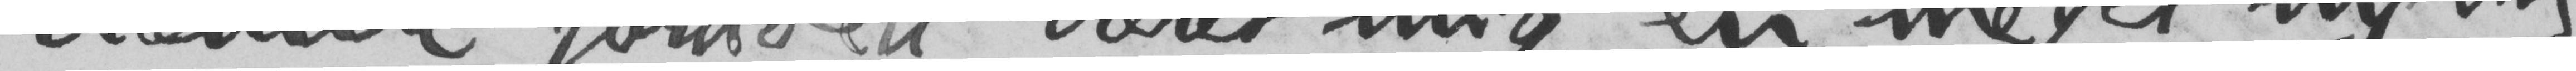nævnte forhold været mig en meget nyttig
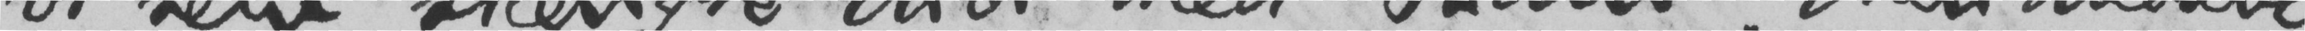vi selv slængte did med Skam». Men allerede
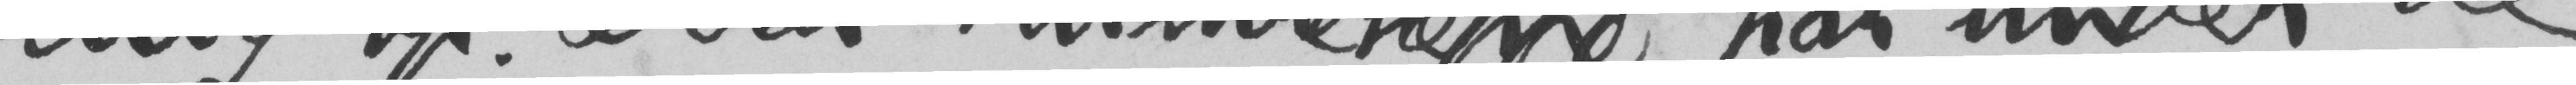mig op. Deres slumretæppe har under de
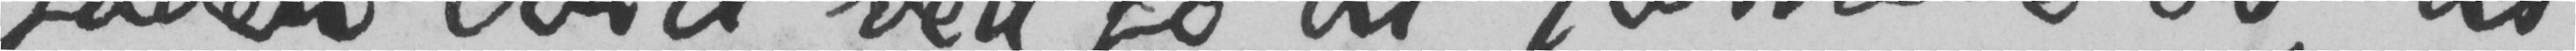fader Ovid ved jo at fortælle os, at
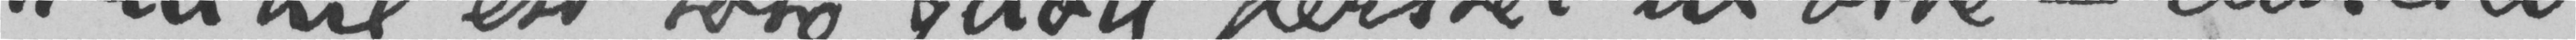«nihil est toto quod perstet in orbe – cuncta
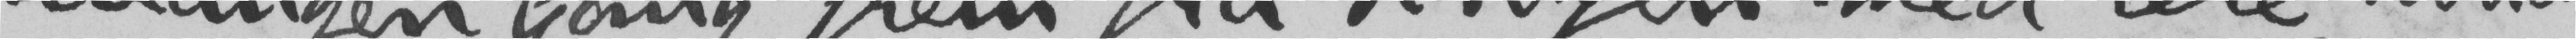mangen Gang frem fra Krogen med Ære, hvad
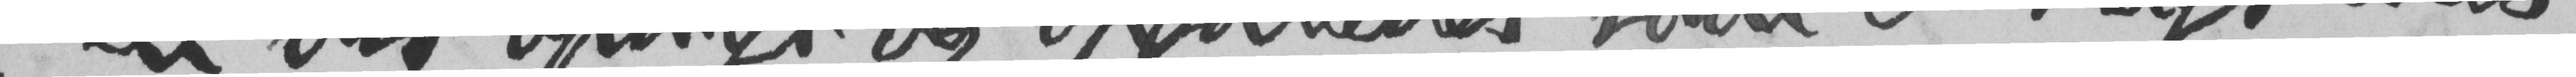en vis opsigt og opfattedes som et slags æres-
